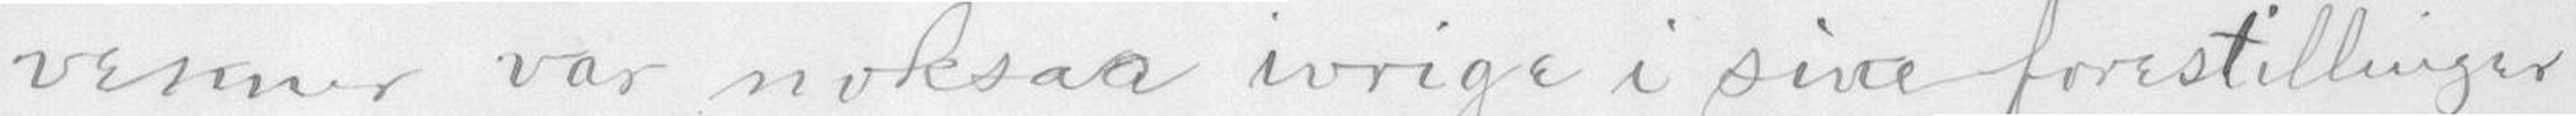venner var noksaa ivrige i sine forestillinger
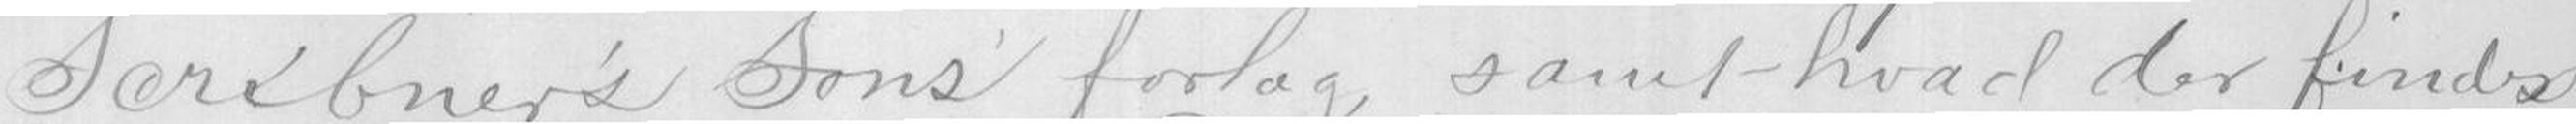Screbner's Son's forlag, samt-hvad der findes
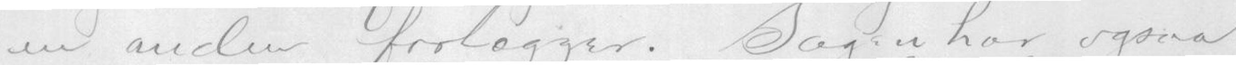en anden forlegger. Sagen har ogsaa
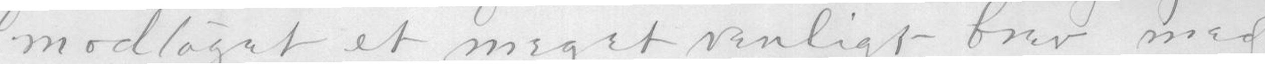modtaget et meget venligt brev med
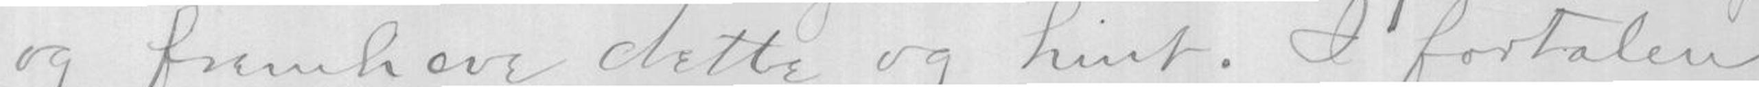og fremheve dette og hint. I fortalen
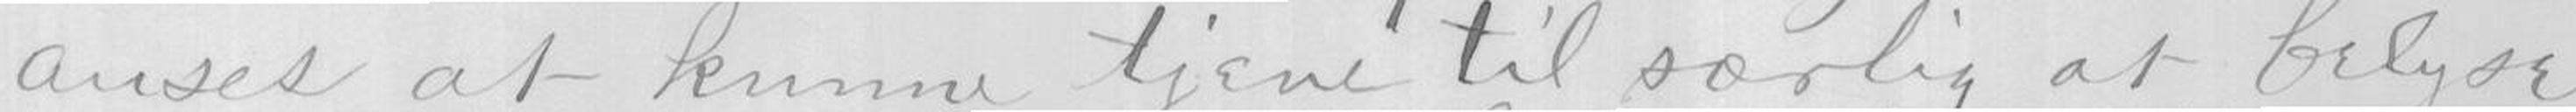anses at kunne tjene til særlig at belyse
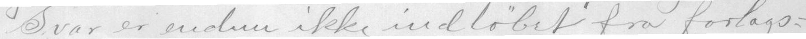Svar er endnu ikke indløbet fra forlags-
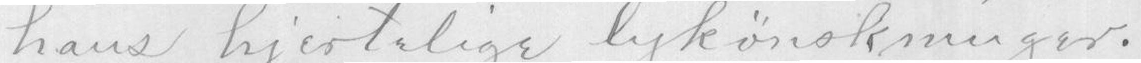hans hjertelige lykønskninger.
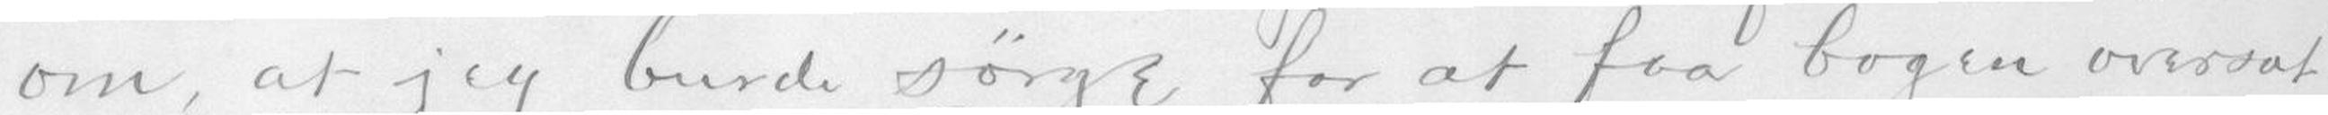om, at jeg burde sørge forat faa bogen oversat
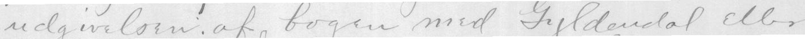udgivelsen af bogen med Gyldendal eller
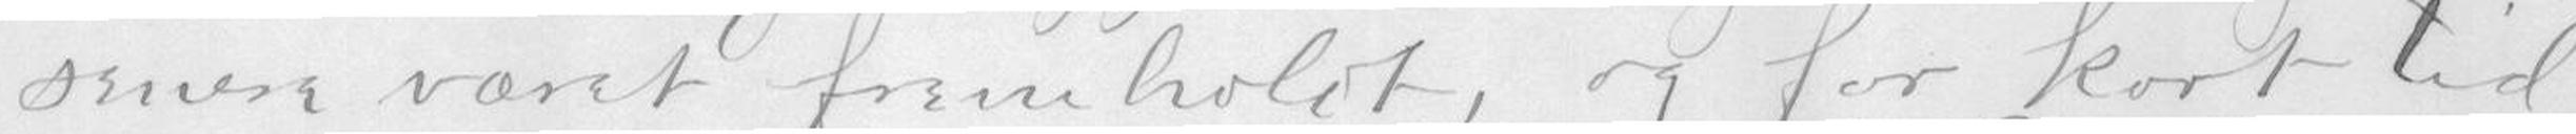senere været fremholdt, og for kort tid
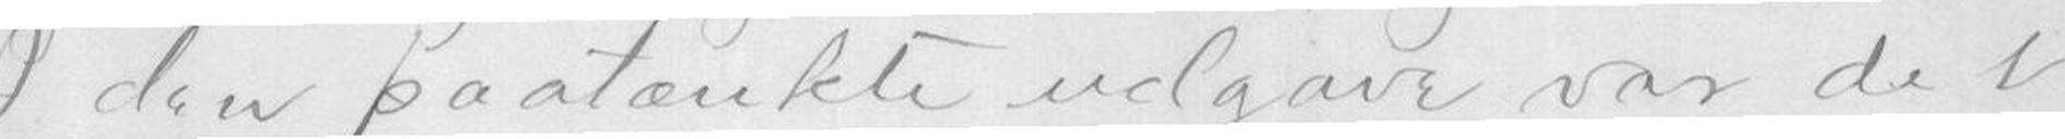I den paatænkte udgave var det
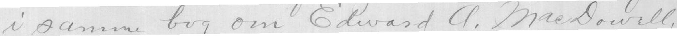i samme bog om Edvard A. MacDowell,
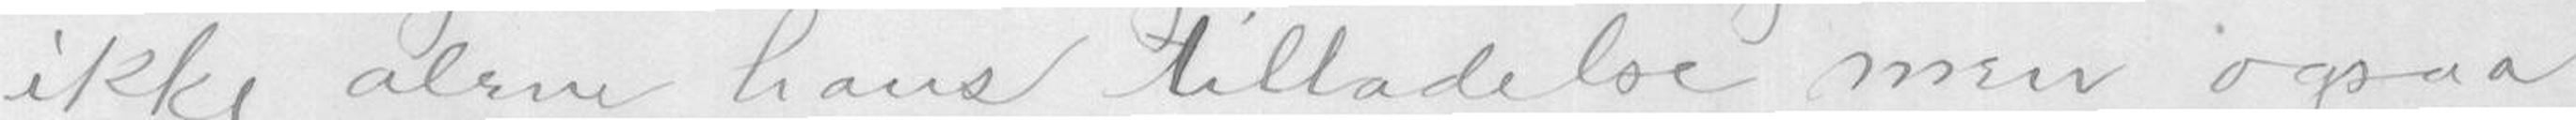ikke alene hans tilladelse, men ogsaa
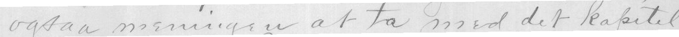ogsaa meningen at ta med det kapitel
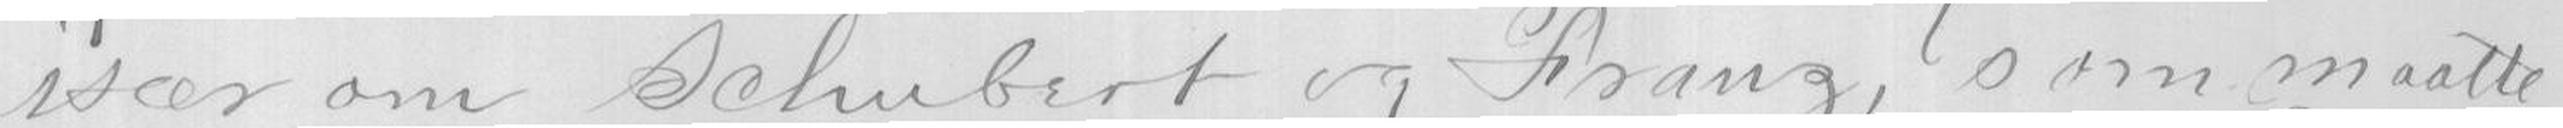især om Schubert og Franz, som maatte
